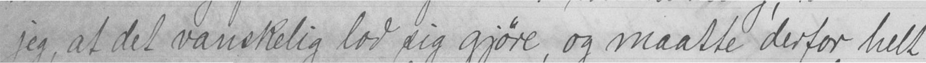jeg, at det vanskelig lod sig gjøre, og maatte derfor helt
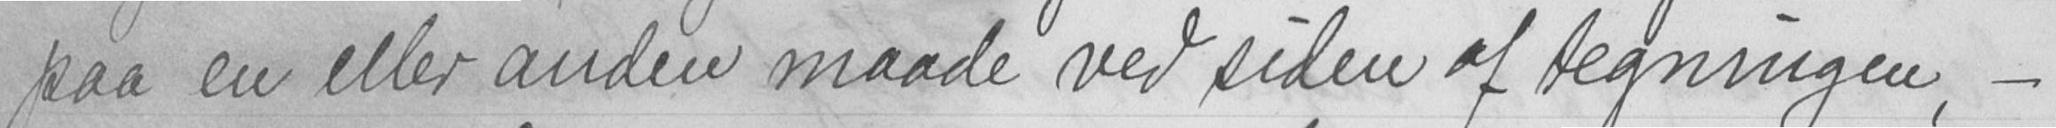paa en eller anden maade ved siden af tegningen, -
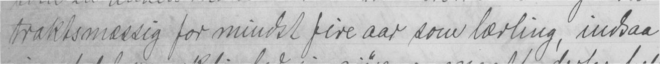traktsmæssig for mindst fire aar som lærling, indsaa
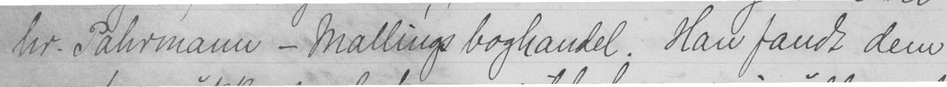hr. Pahrmann - Mallings boghandel. Han fandt dem
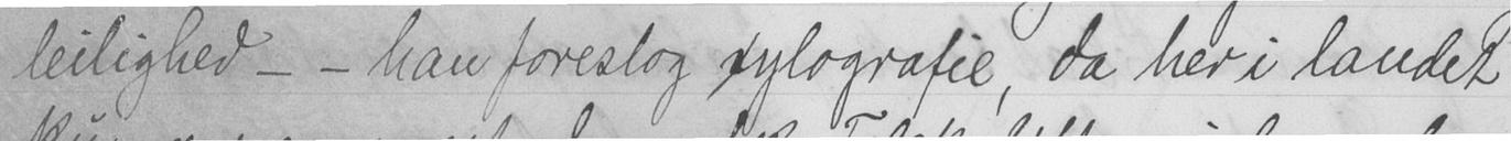leilighed - - han foreslog sylografie, da her i landet
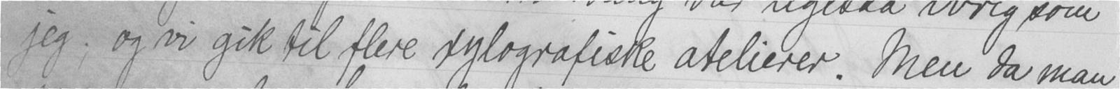jeg; og vi gik til flere sylografiske atelierer. Men da man
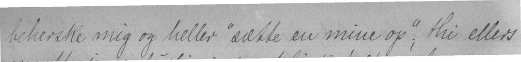beherske mig og heller "sætte en mine op"; thi ellers
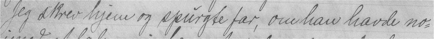Jeg skrev hjem og spurgte far, om han havde no-
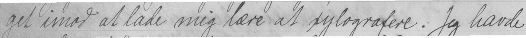get imod at lade mig lære at sylografere. Jeg havde
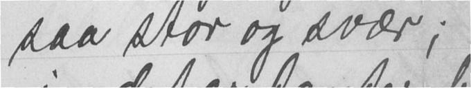saa stor og svær;
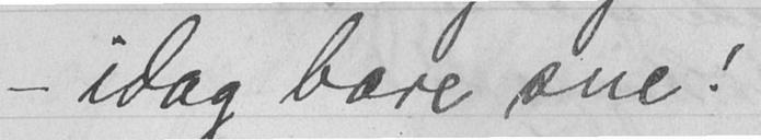- idag bare sne!
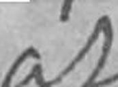at
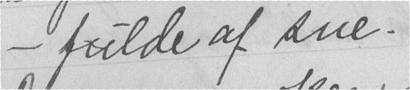- fulde af sne.
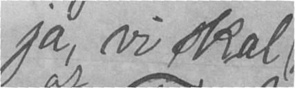ja, vi skal
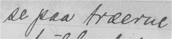se paa træerne
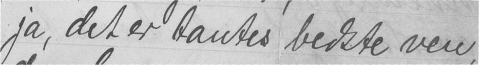ja, det er tantes bedste ven,
